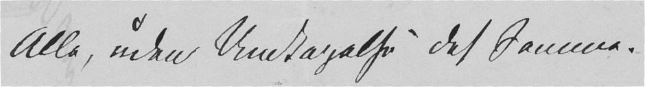Alle, uden Undtagelse det Samme.
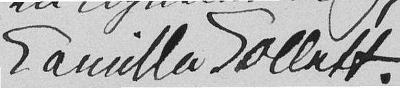Camilla Collett
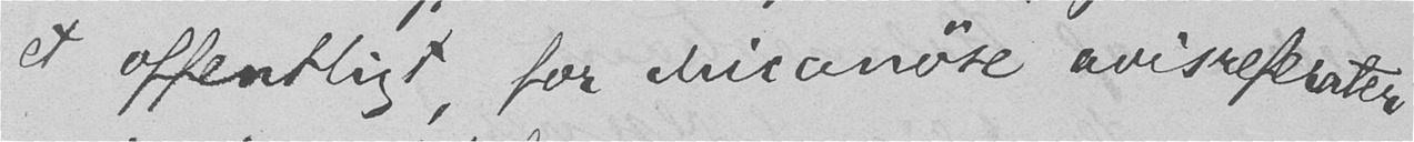et offentligt, for chicanøse avisreferater
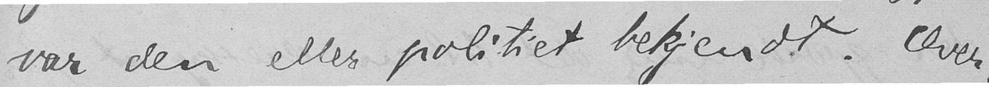var den eller politiet bekjendt. Over-
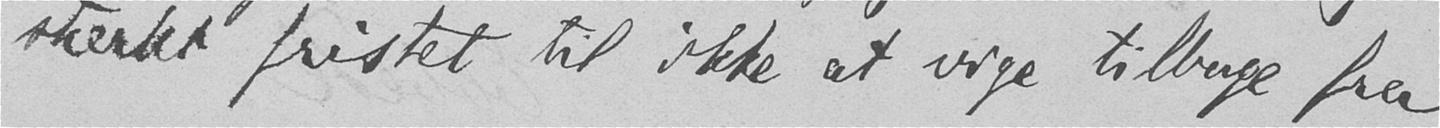stærkt fristet til ikke at vige tilbage fra
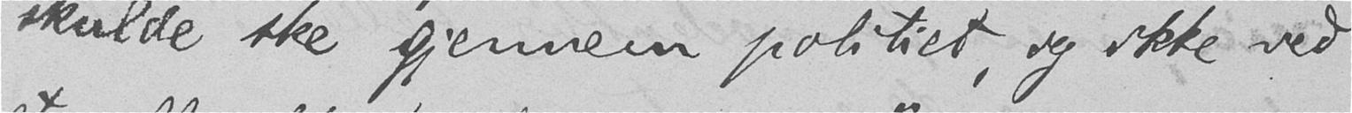skulde ske gjennem politiet, og ikke ved
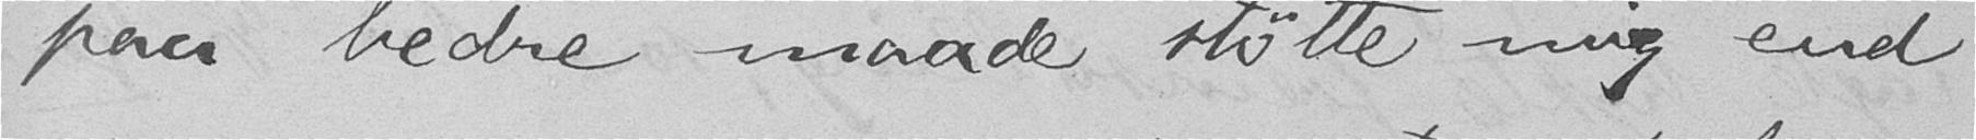paa bedre maade støtte mig end
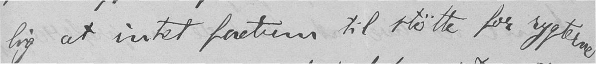lig at intet factum til støtte for rygterne
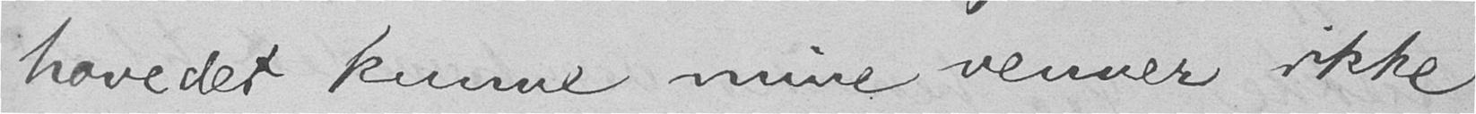hovedet kunne mine venner ikke
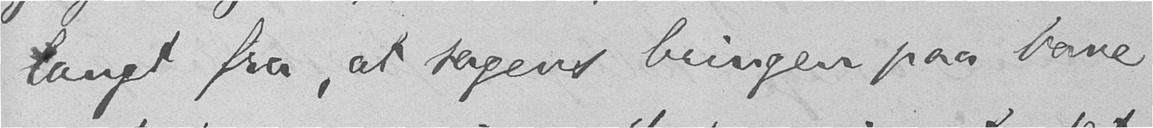langt fra, at sagens bringen paa bane
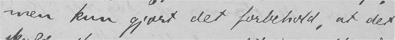men kun gjort det forbehold, at det
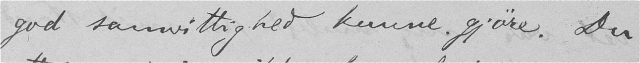god samvittighed kunne gjøre. Du
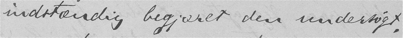indstændig begjæret den undersøgt,
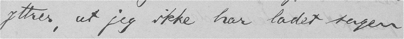yttrer, at jeg ikke har ladet sagen
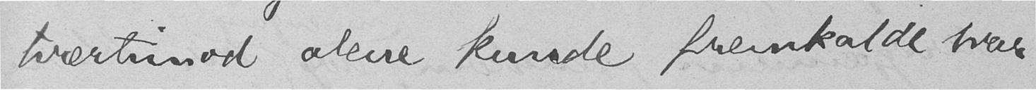tværtimod alene kunde fremkalde svar
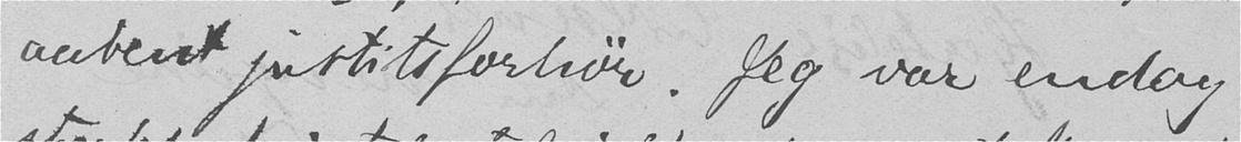aabent justitsforhør. Jeg var endog
